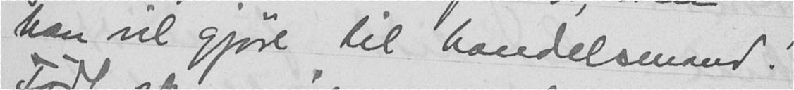han vil gjøre til handelsmand!
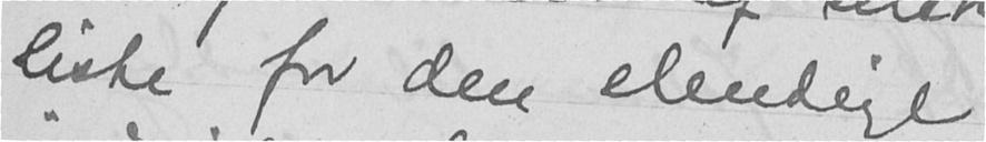liste for den elendige
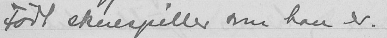Født skuespiller som han er.
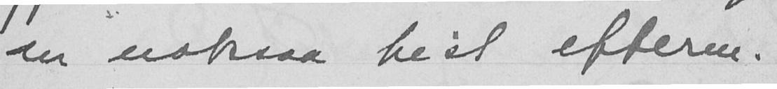en noksaa trist efterm.
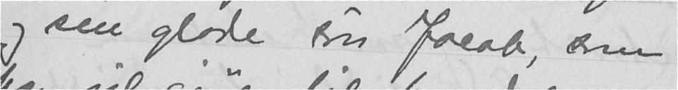og sin glade søn Jacob, som
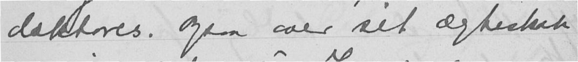doktorer. Ogsaa over sit ægteskab
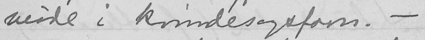møde i kvindesagsforn. -
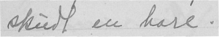skudt en hare.
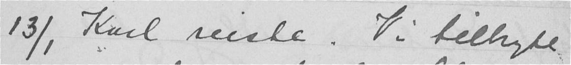13/1 Karl reiste. Vi tilbragte
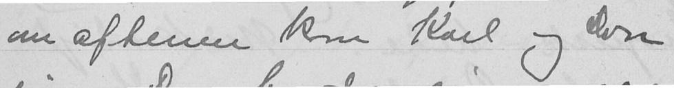om aftenen kom Karl og Don
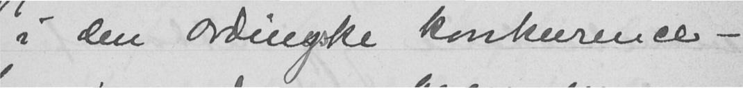i den ordingske konkurence -
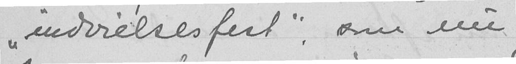"indvielsesfest", som nu
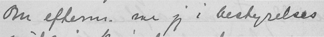Om efterm. var jeg i bestyrelses
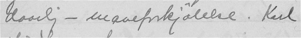daarlig - maveforkjølelse. Karl
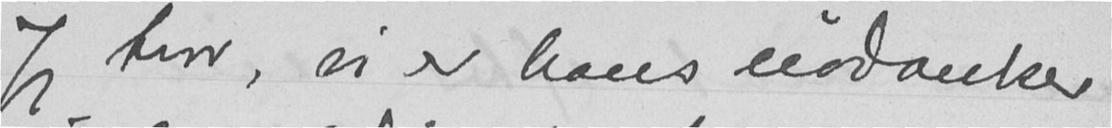Jeg tror, vi er hans nødanker
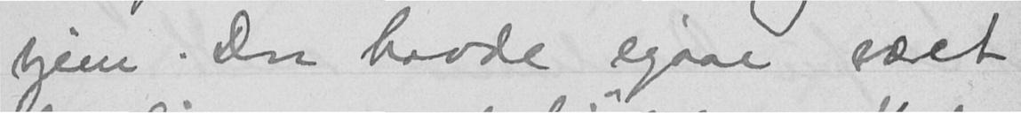hjem. Don havde igaar været
